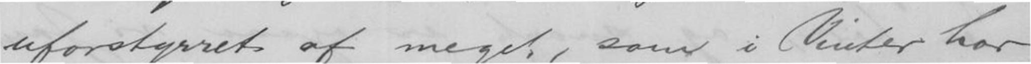uforstyrret af meget, som i Vinter har
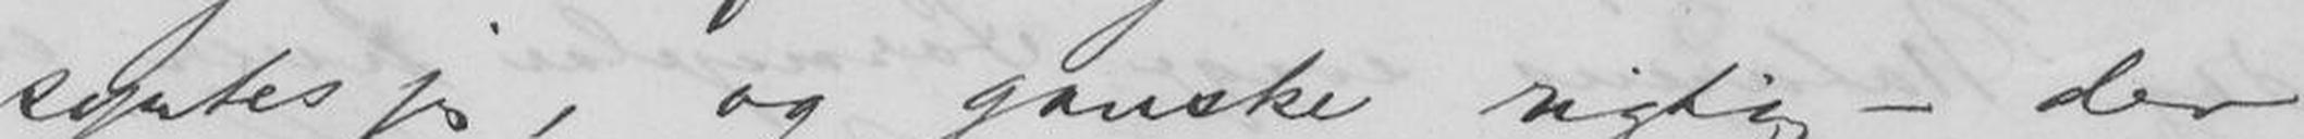syntes jeg, og ganske rigtig - der
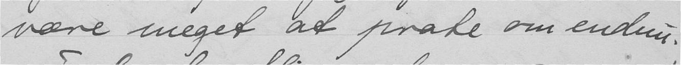være meget at prate om endnu.
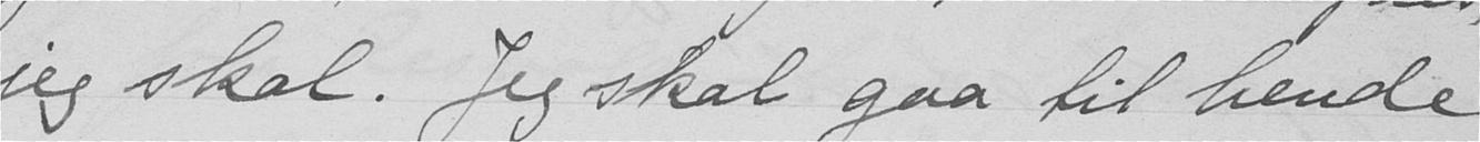jeg skal. Jeg skal gaa til hende
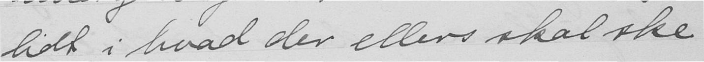lidt i hvad der ellers skal ske
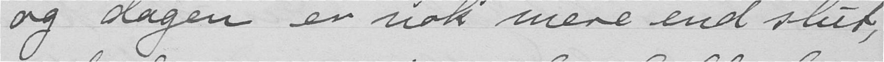og dagen er nok mere end slut,
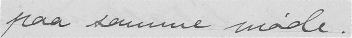paa samme møde.
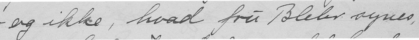- og ikke, hvad fru Bleler synes,
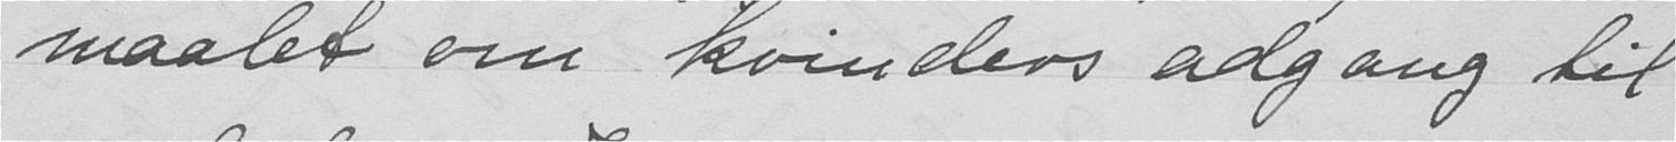maalet om kvinders adgang til
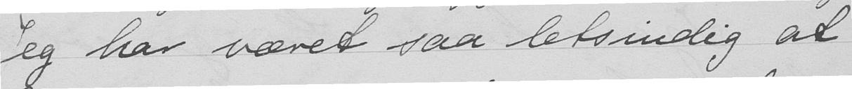Jeg har været saa letsindig at
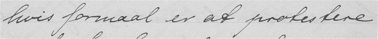hvis formaal er at protestere
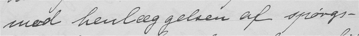mod henlæggelsen af spørgs-
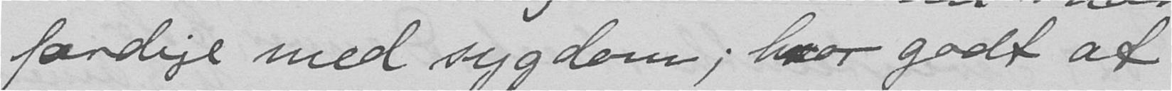færdige med sygdom; hvor godt at
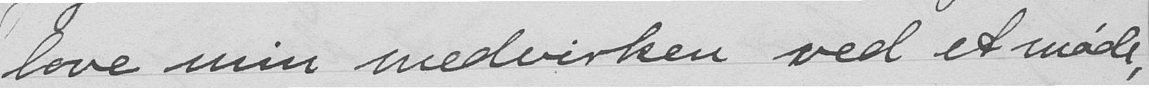love min medvirken ved et møde,
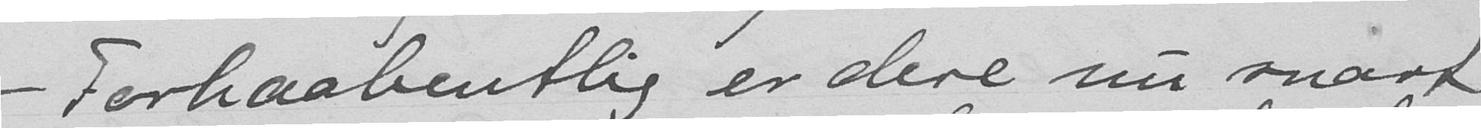- Forhaabentlig er dere nu snart
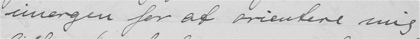imorgen for at orientere mig
